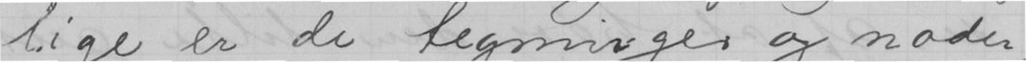lige er de tegninger og noder
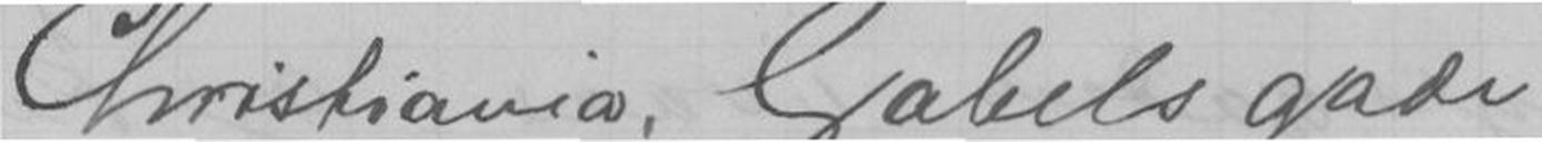Christiania,Gabelsgade
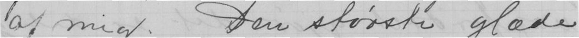af mig. Den største glæde
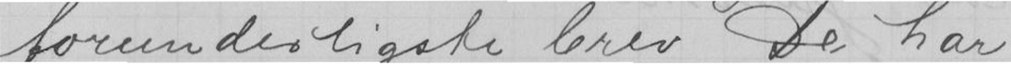forunderligste brev. De har
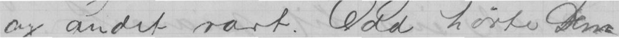og andet rart. Odd hørte Dem
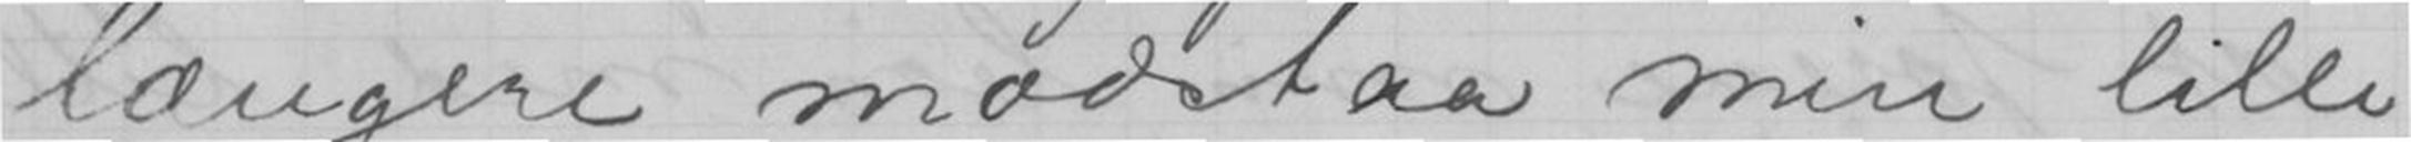længere modstaa min lille
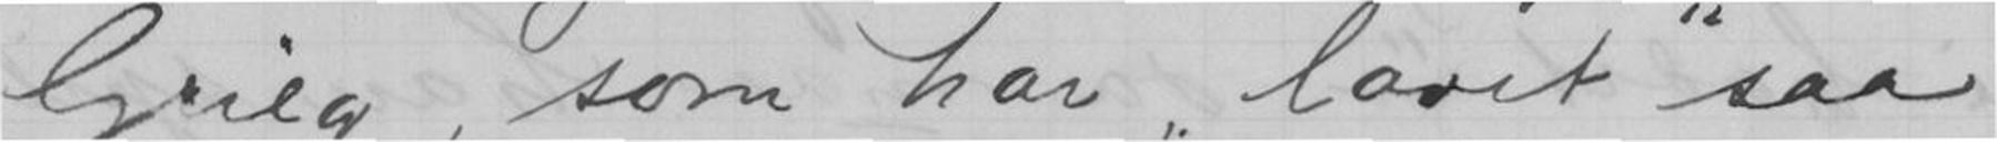Grieg, som har "lovet" saa
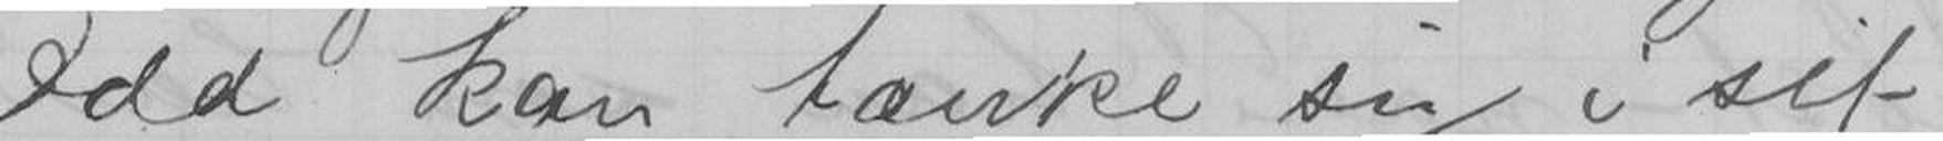Odd kan tænke sig i sit
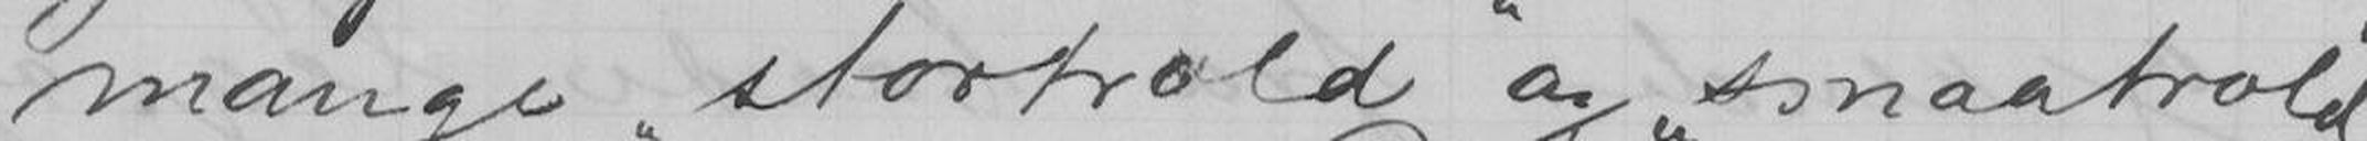mange stortrold og smaatrold
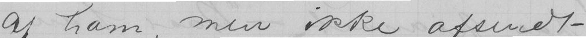af ham, men ikke afsendt -
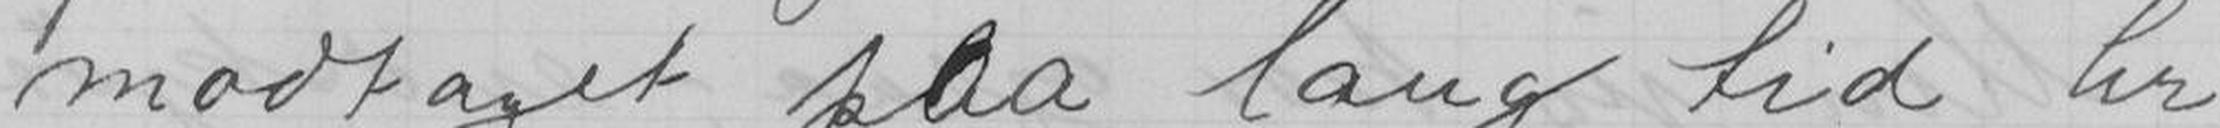modtaget paa lang tid. hr.
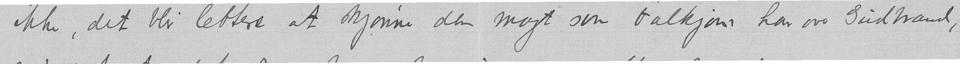ikke, det blir lettere at skjønne den magt som Falkjom har over Gudbrand,
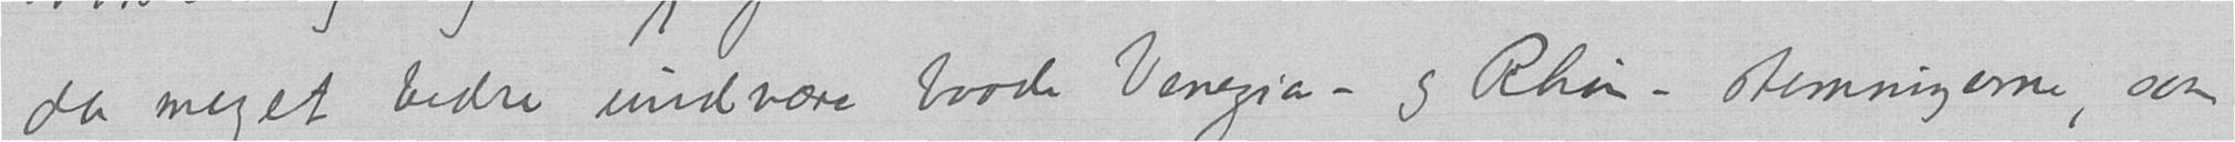da meget bedre undvære baade Venezia – og Rhin-stemningerne, som
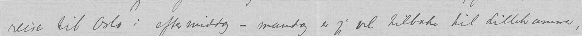reise til Oslo i eftermiddag – mandag er jeg vel tilbake til Lillehammer,
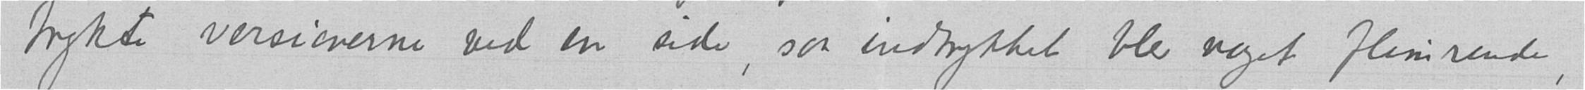trykte versionerne ved en side, saa indtrykket blev noget flimrende,
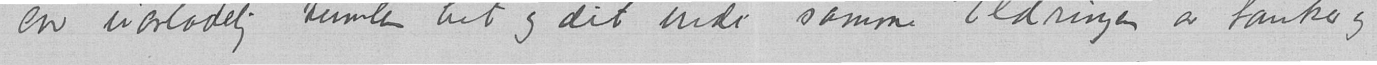en uavladelig tumlen hit og dit indi samme Eldringen av tanker og
